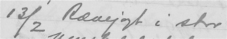13/2 Rævejagt i stor
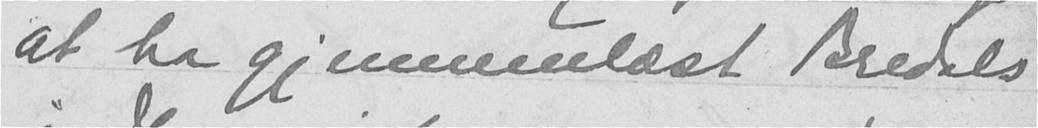at ha gjennemlæst Bredals
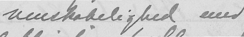venskabelighed med
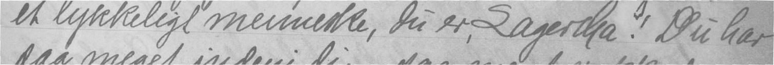et lykkeligt menneske, du er, Lagertha! Du har
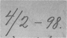4/2-98.
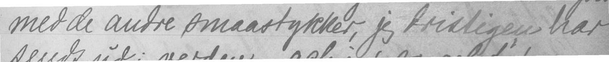med de andre smaastykker, jeg dristigen har
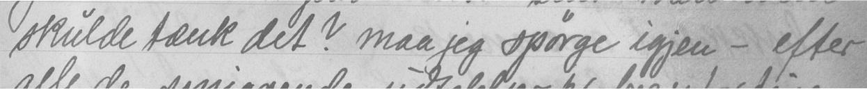skulde tænk det? maa jeg spørge igjen - efter
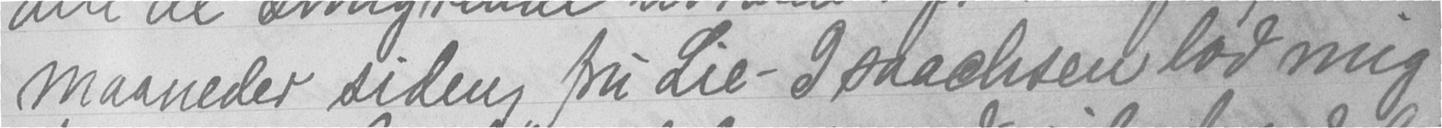maaneder siden, fru Lie-Issachsen lod mig
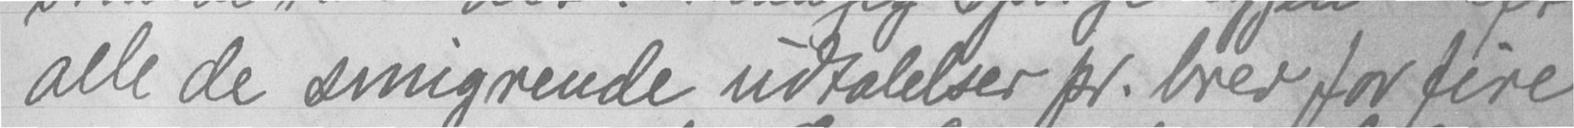alle de smigrende udtalelser pr. brev for fire
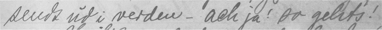sendt ud i verden - ach ja! so gelt's!
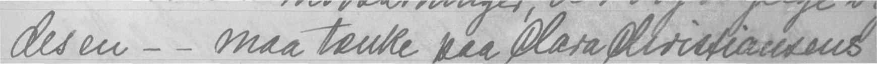des en - - maa tænke paa Clara Ciristiansens
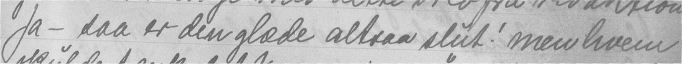Ja - saa er den glæde altsaa slut! men hvem
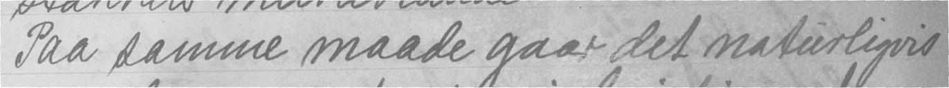Paa samme maade gaar det naturligvis
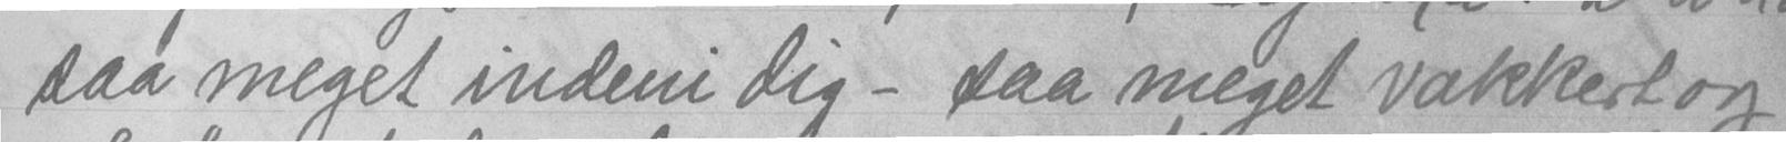saa meget indeni dig - saa meget vakkert og
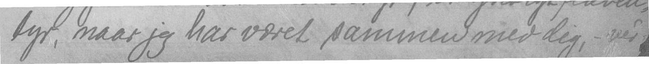tyr, naar jeg har været sammen med dig, - ved
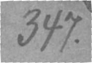347.
